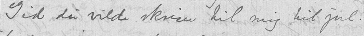Gid du vilde skrive til mig til jul.
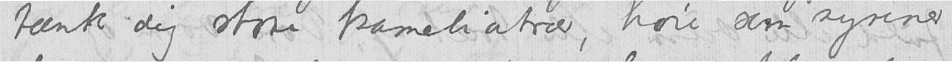tænk dig store kameliatrær, høie som syriner
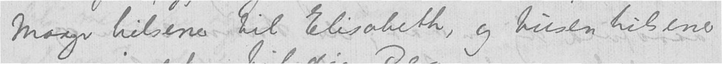Mange hilsener til Elisabeth, og tusen hilsener
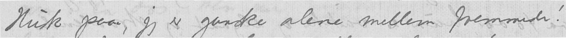Husk paa, jeg er ganske alene mellem fremmede!
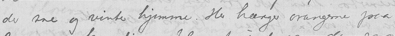der sne og vinter hjemme. Her hænger orangerne paa
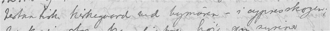testantiske kirkegaard ved bymuren - i cypresskogen;
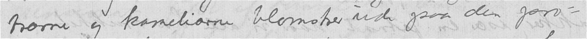trærne og kameliarne blomstrer ude paa den pro-
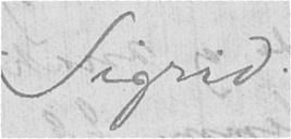Sigrid.
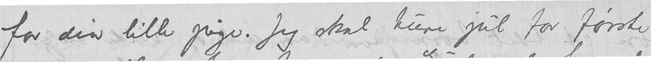for din lille pige. Jeg skal ture jul for første
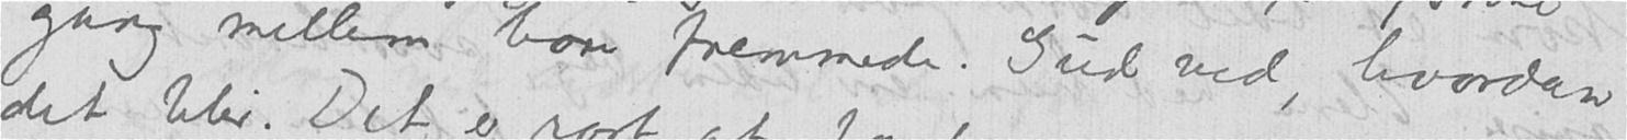gang mellem bare fremmede. Gud ved, hvordan
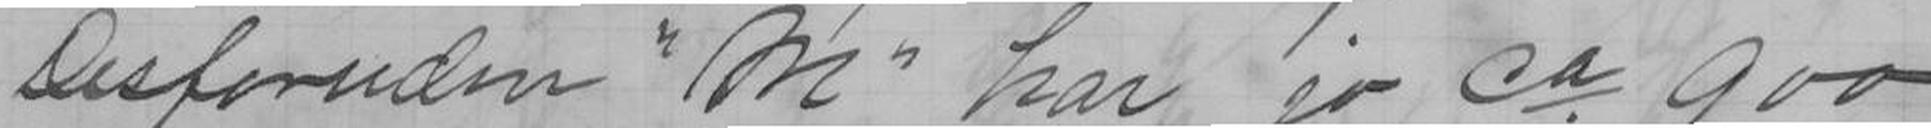Desforuden "M" har jo ca 900
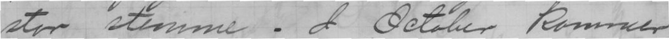stor stemme. I October kommer
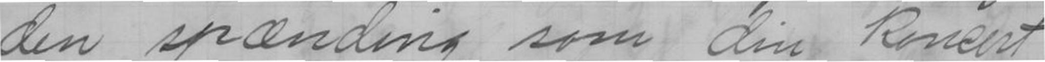den spænding som din koncert
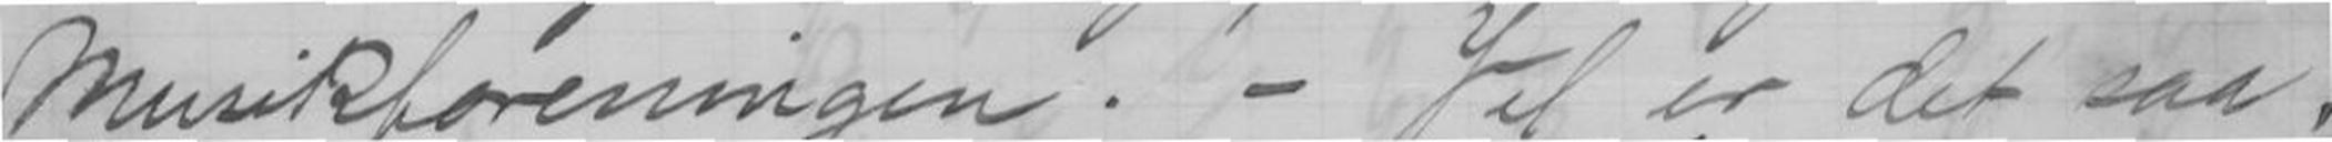Musikforeningen. - Vel er det saa,
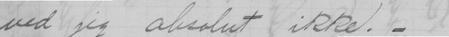ved jeg absolut ikke. -
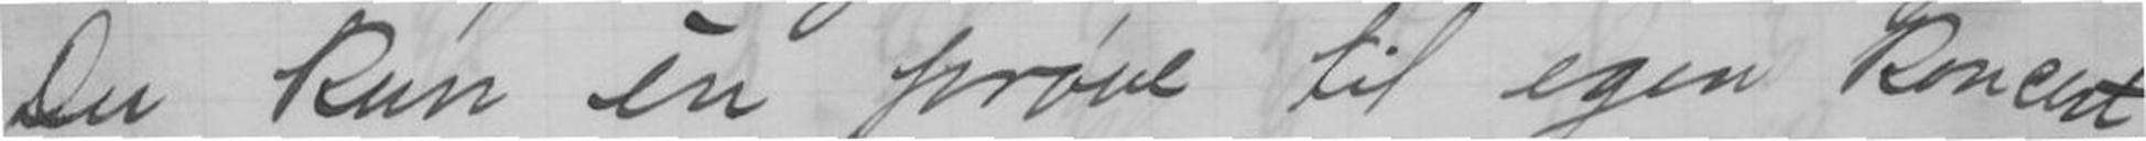Du kun èn prøve til egen koncert,
